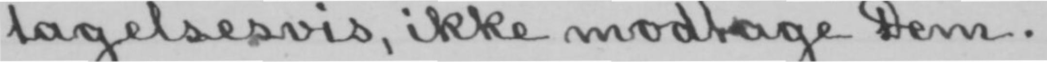tagelsesvis, ikke modtage Dem.
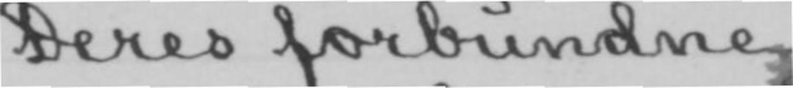Deres forbundne
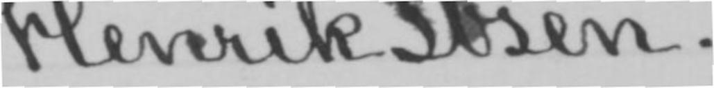Henrik Ibsen.
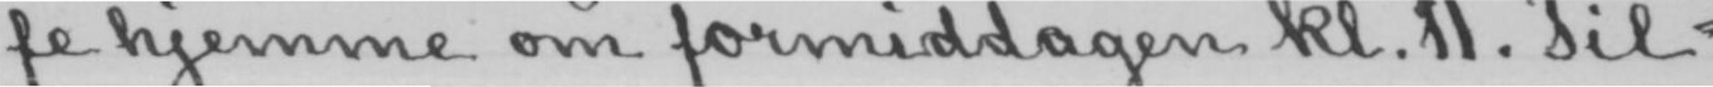fe hjemme om formiddagen kl. 11. Til-
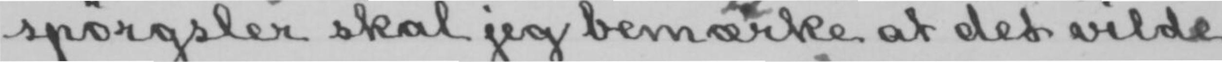spørgsler skal jeg bemærke at det vilde
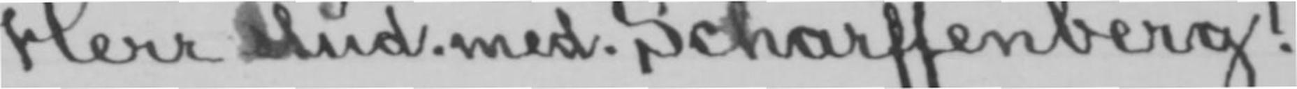Herr stud. med. Scharffenberg!
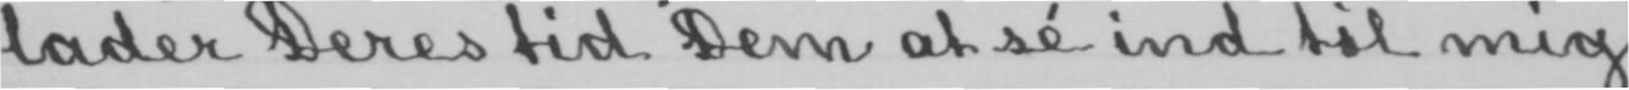lader Deres tid Dem at sé ind til mig
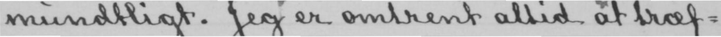mundtligt. Jeg er omtrent altid at træf-
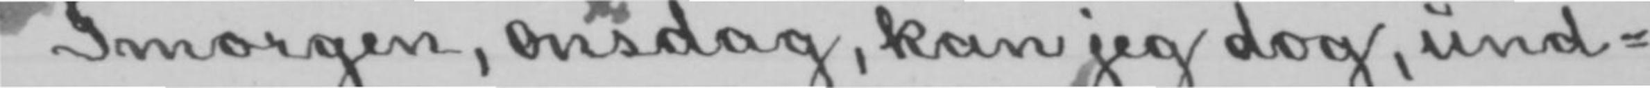Imorgen, onsdag, kan jeg dog, und-
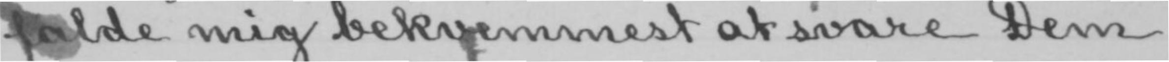falde mig bekvemmest at svare Dem
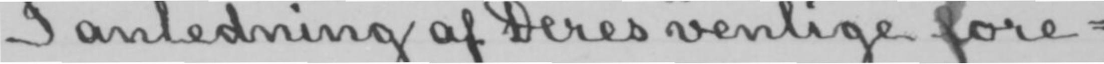I anledning af Deres venlige fore-
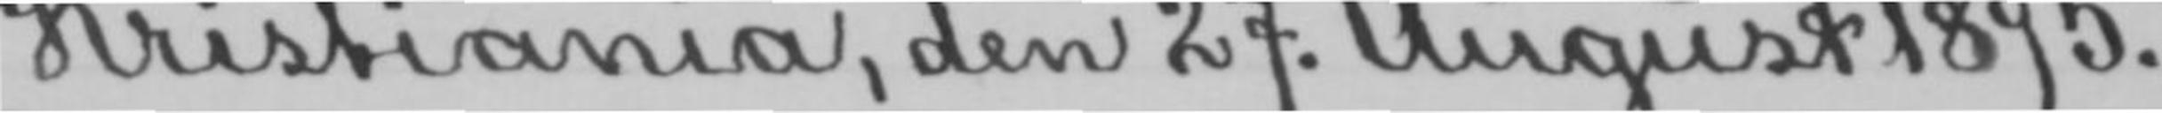Kristiania, den 27. August 1895.
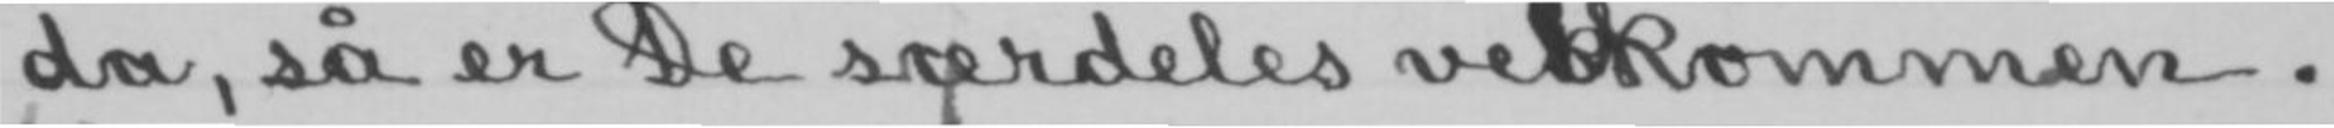da, så er De særdeles veklkommen.
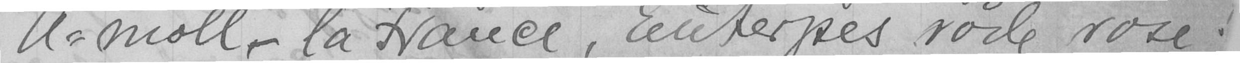A-moll - la France, Euterpes røde rose!
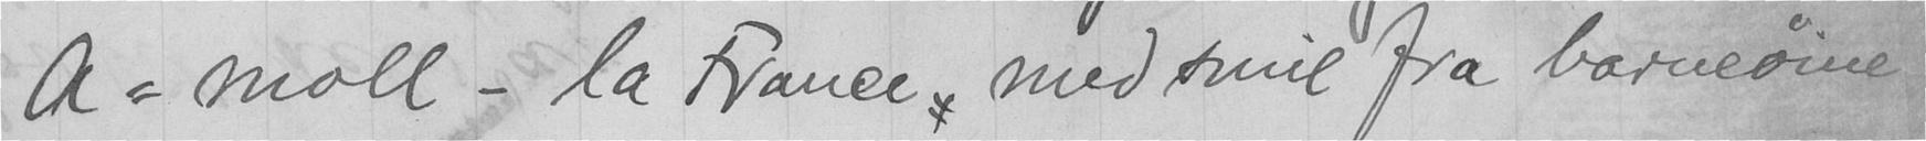A-moll - la France med smil fra barneøine
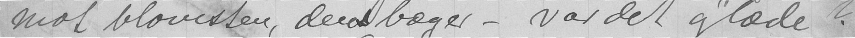mot blomsten, den bæger - var det glæde?
